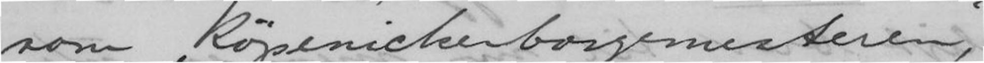som "Köpenickerborgermesteren",
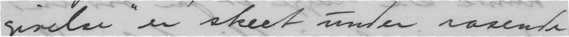givelse" er skeet under rasende
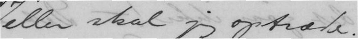eller skal jeg optræde!
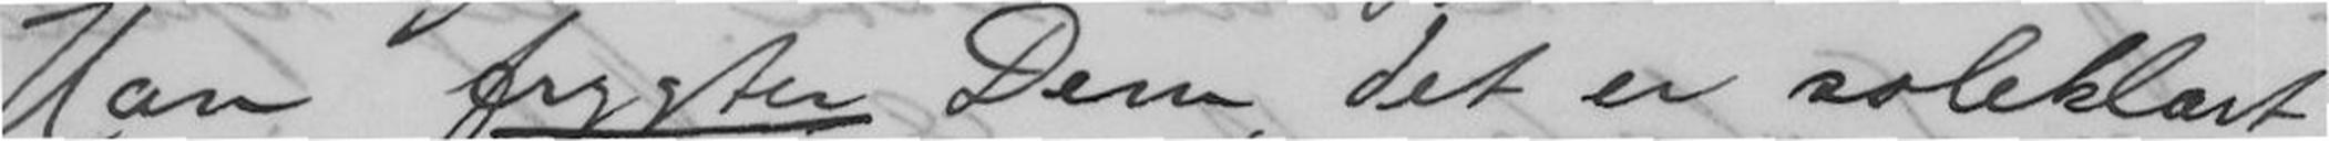Han frygter Dem, det er soleklart,
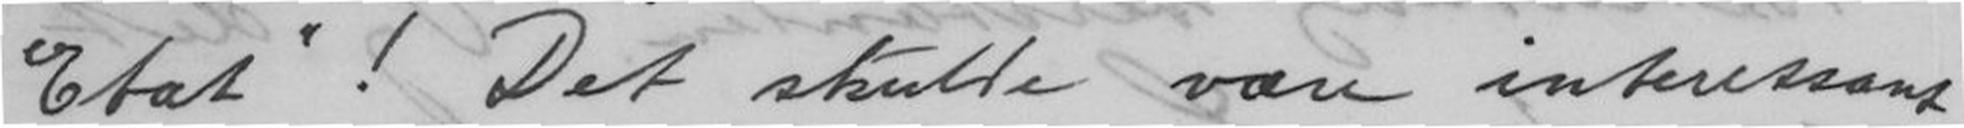Etat"! Det skulde være interessant
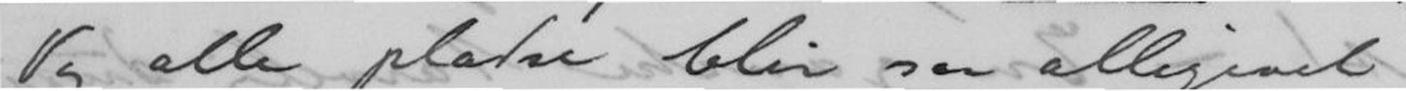Og alle pladse blir saa alligevel
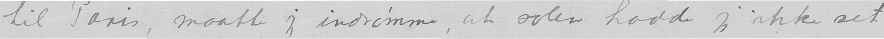til Paris, maatte jeg indrømme, at solen hadde jeg ikke set,
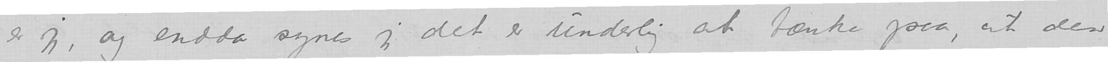og endda synes jeg det er underlig at tænke paa, at den
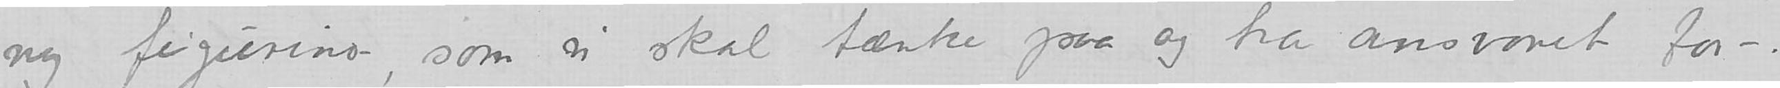ny figurino, som vi skal tænke paa og ha ansvaret for-
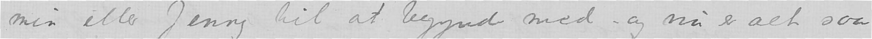min eller Jenny til at begynde med - og nu er det saa
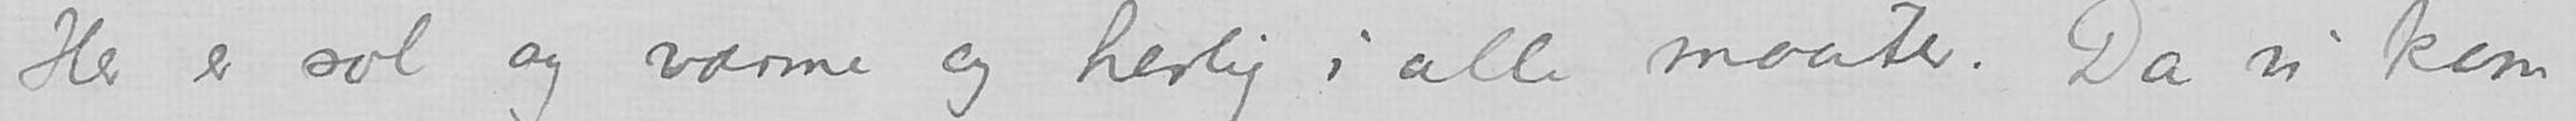Her er sol og varme og herlig i alle maater. Da vi kom
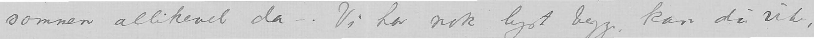sammen allikevel da -. Vi har nok lyst begge, kan du vite,
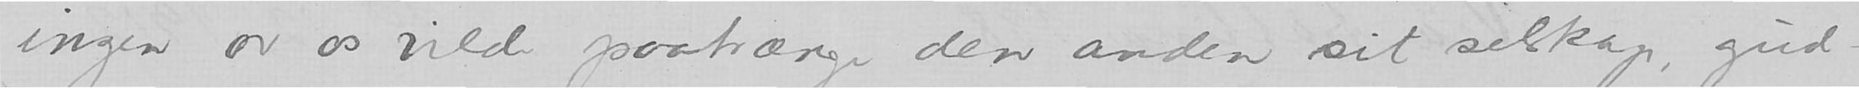ingen av os vilde paatrænge den anden sit selskap, gud
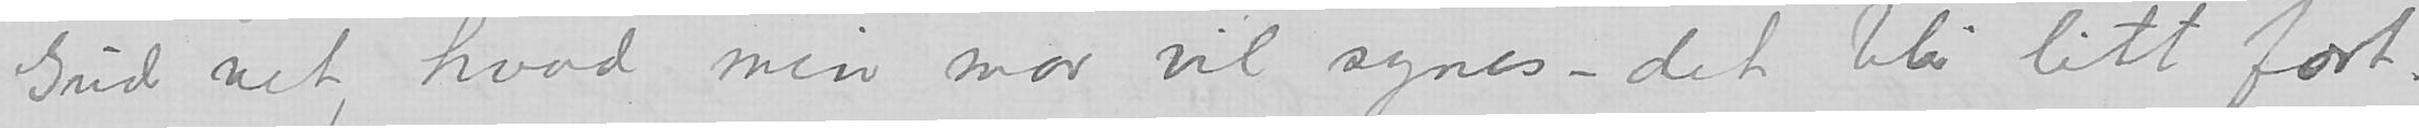Gud vet, hvad min mor vil synes – det blir litt fort.
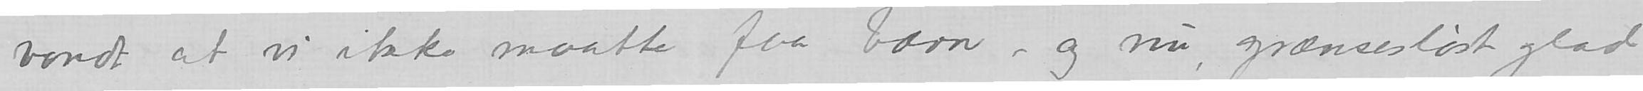vondt at vi ikke maatte faa barn, og nu, grænseløst glad er jeg
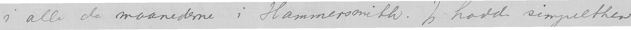i alle de maanederne i Hammersmith. Jeg hadde simpelthen
